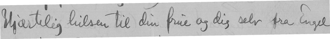Hjærtelig hilsen til din frue og dig selv fra Engel
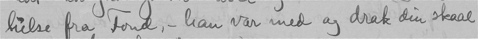hilse fra Fond, - han var med og drak din skaal
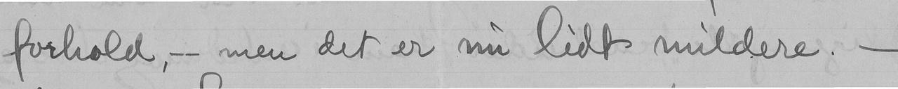forhold, - men det er nu lidt mildere. -
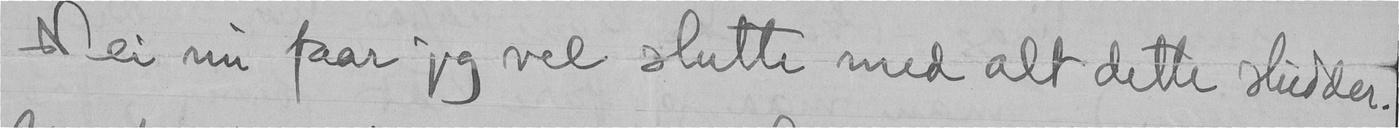Nei nu faar jeg vel slutte med alt dette sludder.
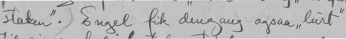staken". Engel fik dengang ogsaa "lurt"
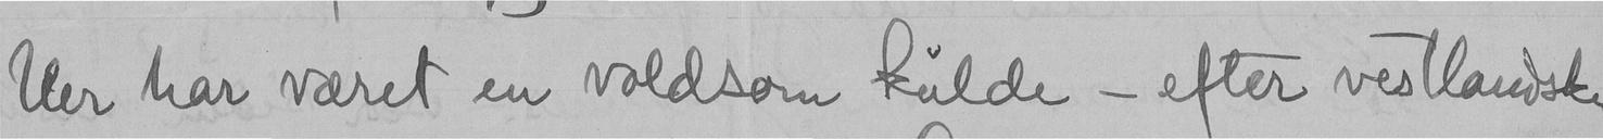Her har været en voldsom kulde - efter vestlandske
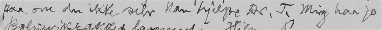paa om du ikke selv kan hjelpe Dr. T. Mig har jo
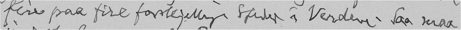fire paa fire forskjellige Steder i Verden. Saa maa
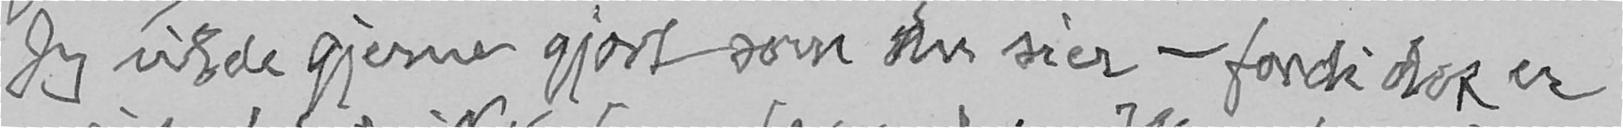Jeg vilde gjerne gjort som du sier - fordi det er
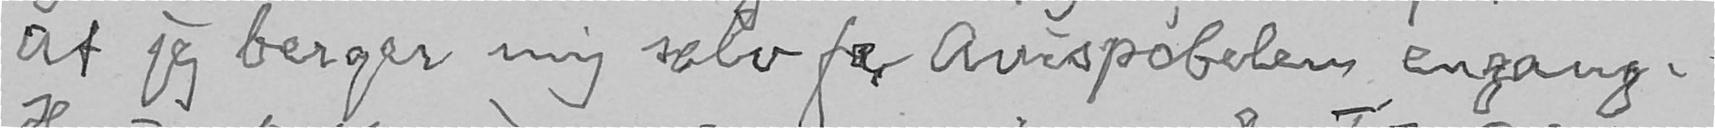at jeg berger mig selv fra Avispøbelen engang.
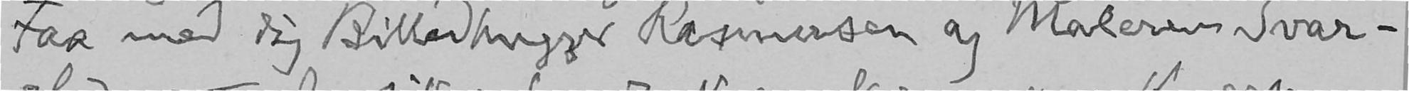Faa med dig Billedhugger Rasmusen og Maleren Svar-
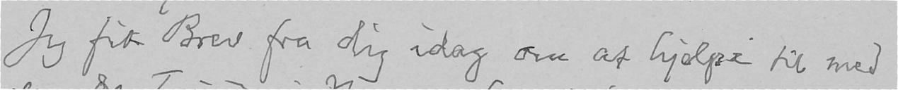Jeg fik Brev fra dig idag om at hjelpe til med
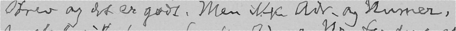Brev og det er godt. Men ikke Adr. og Numer.
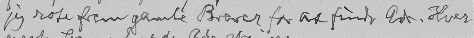jeg rote frem gamle Brever for at finde Adr. Hver
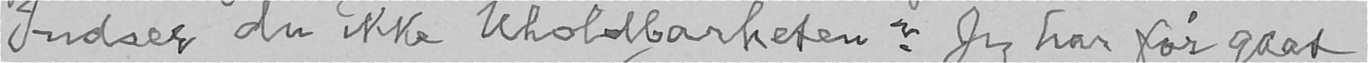Indser du ikke Uholdbarheten? Jeg har før gaat
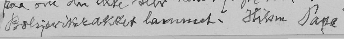Bolsjevikrakket lammet. Hilsen Papa
